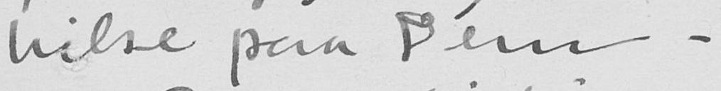hilse paa Dem.
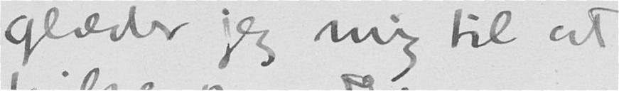glæder jeg mig til at
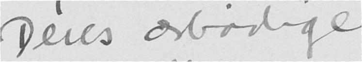Deres ærbødige
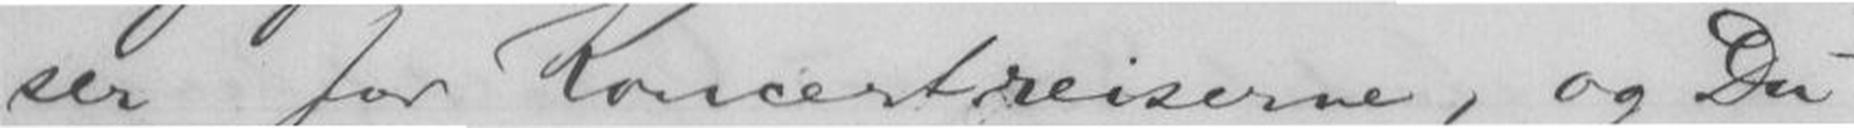ser for Koncertreiserne, og Du
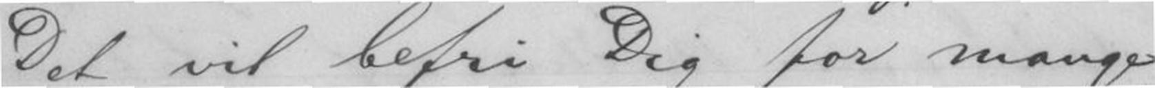Det vil befri Dig for mange
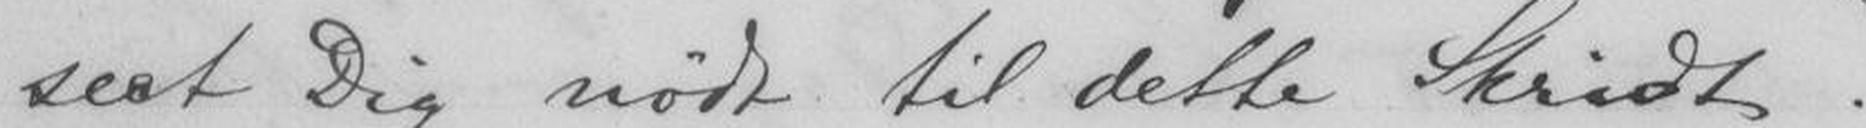seet Dig nødt til dette Skridt.
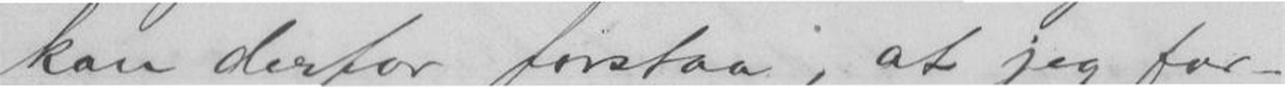kan derfor forstaa, at jeg for-
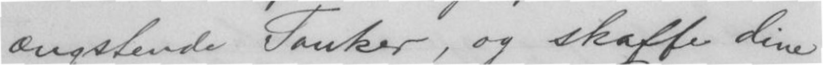ængstende Tanker, og skaffe dine
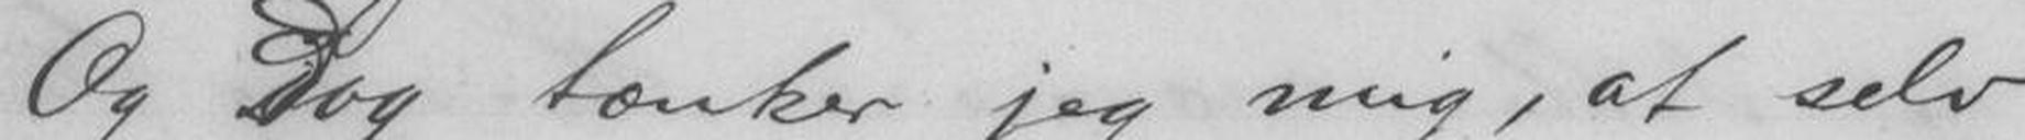Og Dog tænker jeg mig, at selv
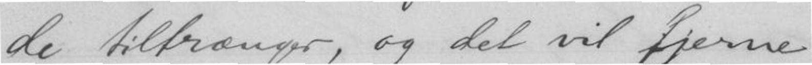de tiltrænger, og det vil fjerne
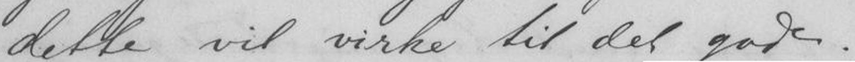dette vil virke til det gode.
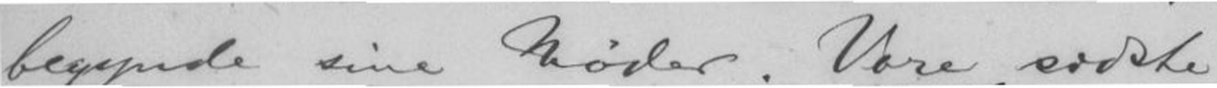begynde sine Møder. Vore sidste
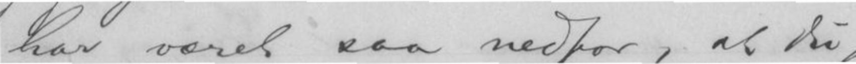har været saa nedfor, at Du
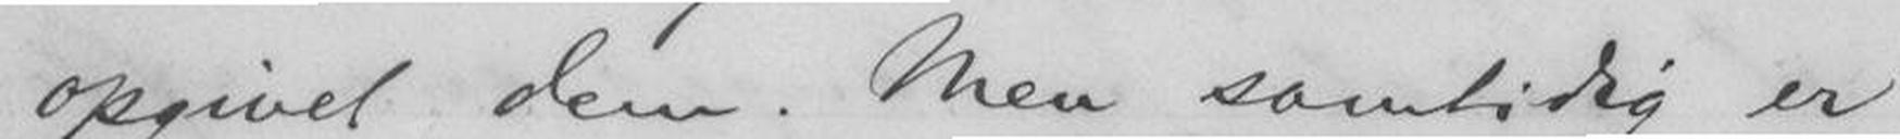opgivet dem. Men samtidig er
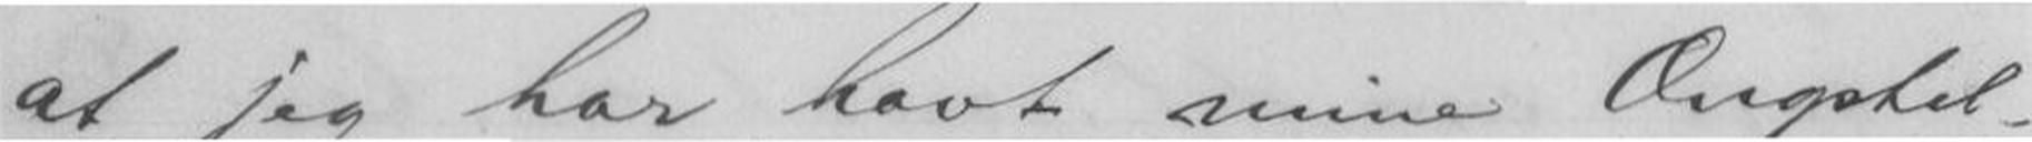at jeg har havt mine Ængstel-
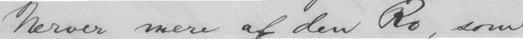Nærver mere af den Ro, som
